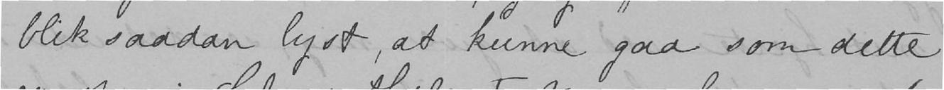blik saadan lyst, at kunne gaa som dette
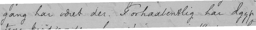gang har været der. Forhaabentlig har Ægyp-
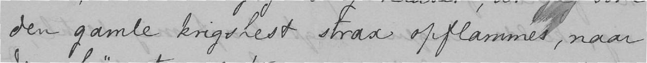den gamle krigshest strax opflammes, naar
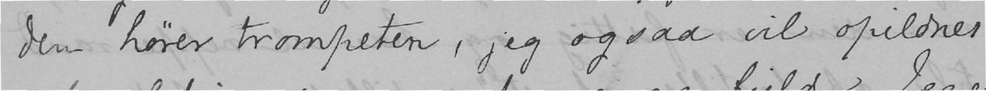den hører trompeten, jeg ogsaa vil opildnes
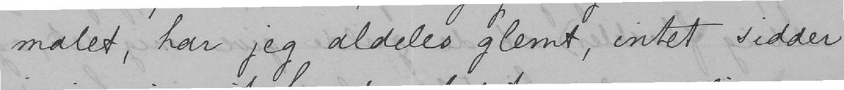malet, har jeg aldeles glemt, intet sidder
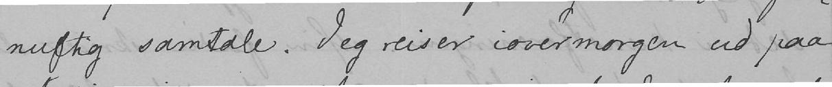nuftig samtale. Jeg reiser iovermorgen ud paa
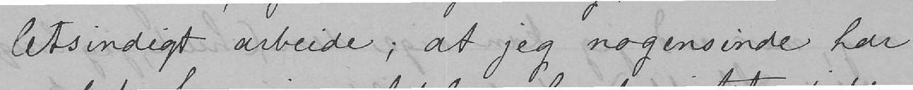letsindigt arbeide; at jeg nogensinde har
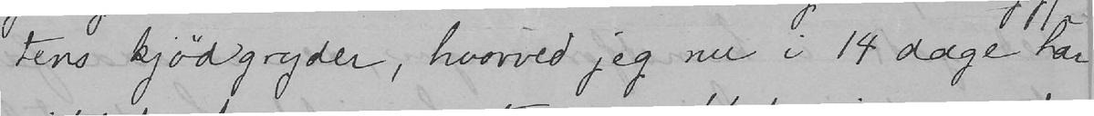tens kjødgryder, hvorved jeg nu i 14 dage har
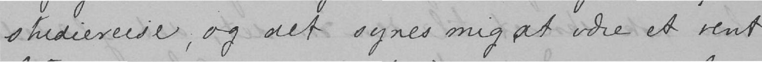studiereise, og det synes mig at være et rent
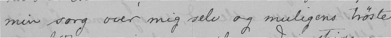min sorg over mig selv og muligens trøste
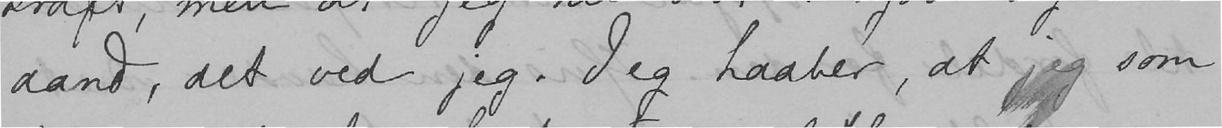aand, det ved jeg. Jeg haaber, at jeg som
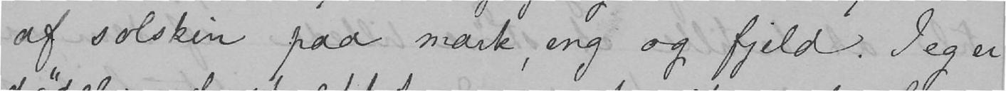af solskin paa mark, eng og fjeld. Jeg er
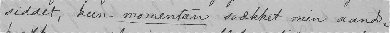siddet, kun momentan svækket min aands
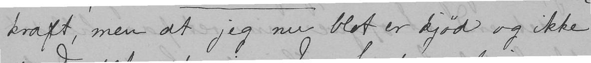kraft, men at jeg nu blot er kjød og ikke
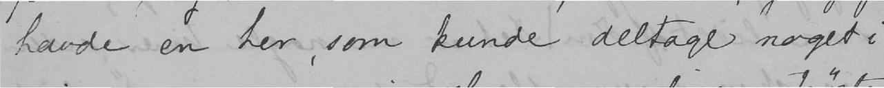havde en her, som kunde deltage noget i
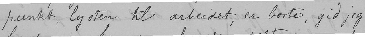punkt, lysten til arbeidet, er borte, gid jeg
